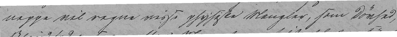neppe vil regne visse physiske Mangler, som Døvhed,
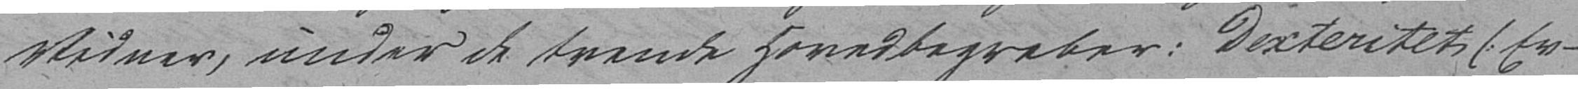Vidner, under de tvende Hovedbegreber: Dexteritet /: Ev-
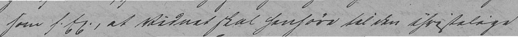som f. Ex., at Vidnet skal henhøre til den christelige
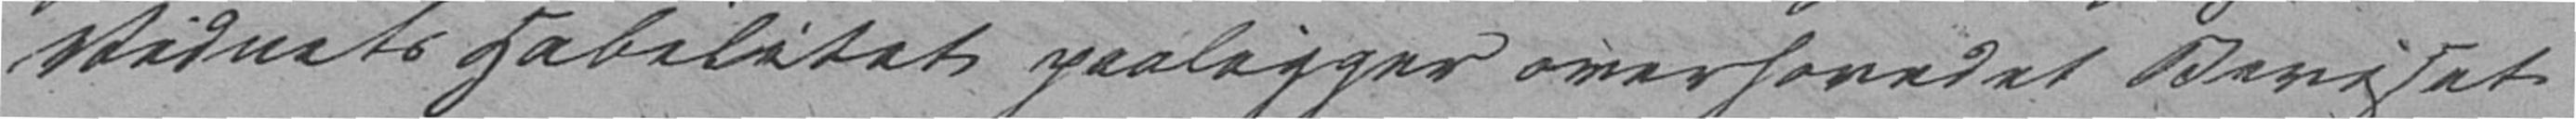Vidnets Habilitet paaligger overhovedet Beviset
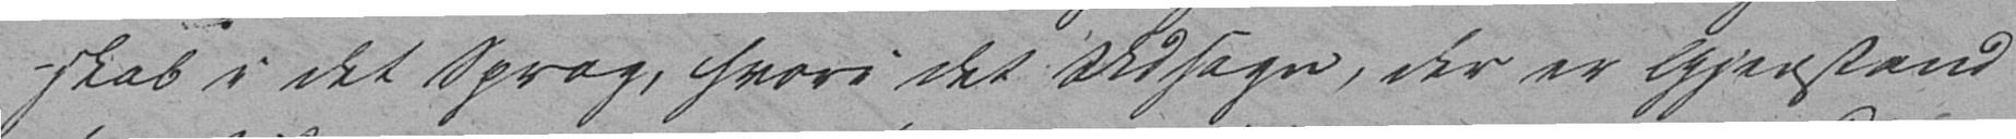skab i det Sprog, hvori det Udsagn, der er Gjenstand
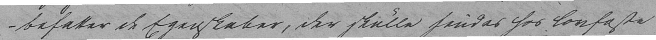befatter de Egenskaber, der skulle findes hos lovfaste
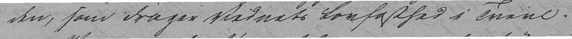den, som drager Vidnets Lovfasthed i Tvivl.
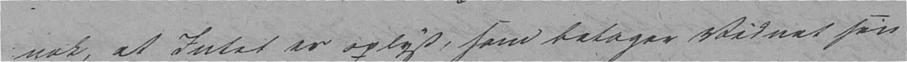nok, at Intet er oplyst, som betager Vidnet sin
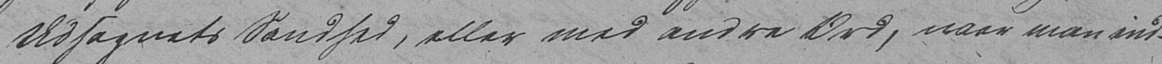Udsagnets Sandhed, eller med andre Ord, naar man ind-
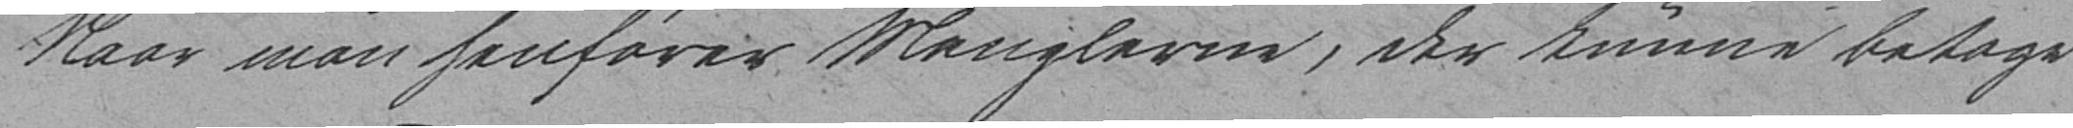Naar man henfører Manglerne, der kunne betage
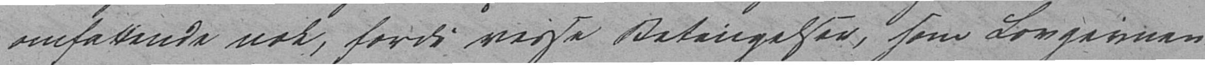omfattende nok, fordi visse Betingelser, som Lovgivnin-
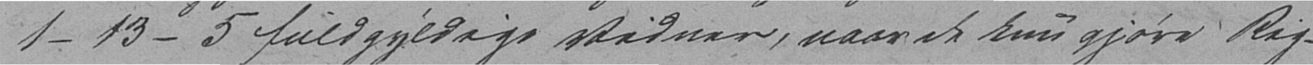1-13-5 fuldgyldige Vidner, naar de kun gjøre Rig-
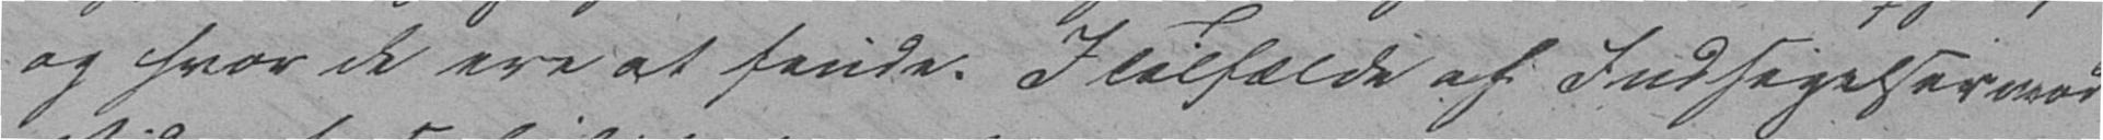og hvor de ere at finde. I Tilfælde af Indsigelser mod
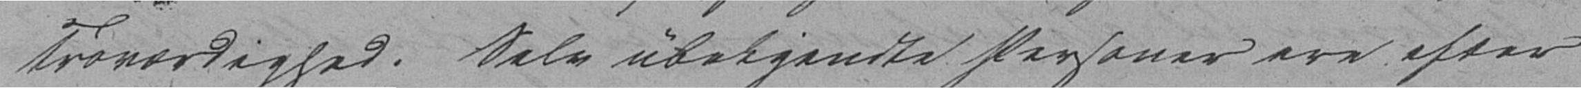Troværdighed. Selv ubekjendte Personer ere efter
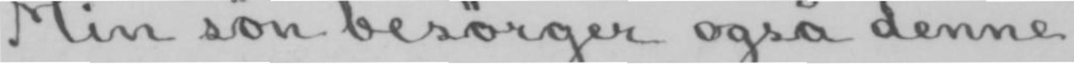Min søn besørger også denne
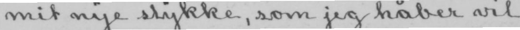mit nye stykke, som jeg håber vil
